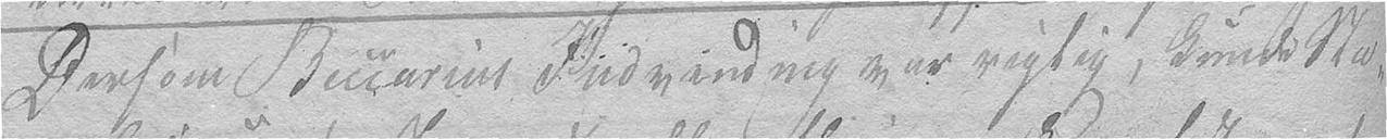Dersom Beccarias Indvending var rigtig, kunde Sta-
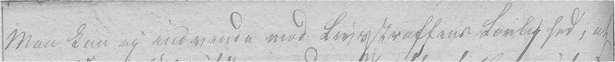Man kan ey indvende mod Livsstraffens Lovlighed, at
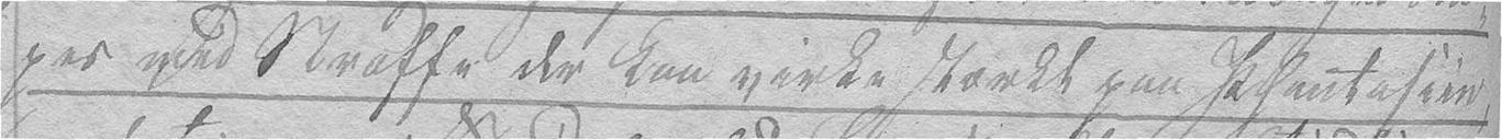ges med Straffe der kan virke stærkt paa Phantasien,
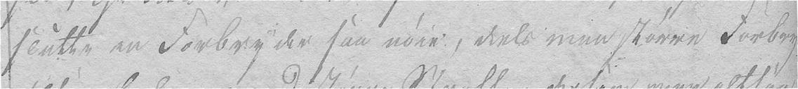slutte en Forbryder saa nøie, deels maa større Forbry-
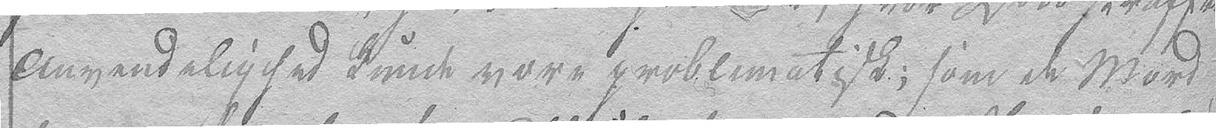Anvendelighed kunde være problematisk, som de Mord
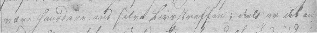være haardere end selve Livsstraffen; deels er det en
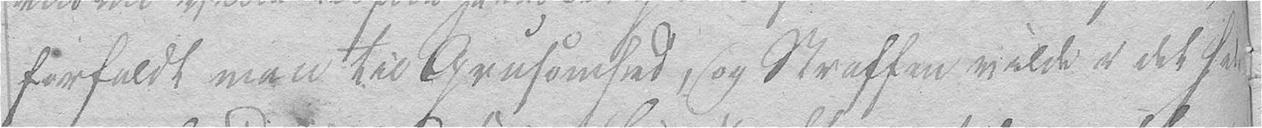forfaldt man til Grusomhed, og Straffen vilde i det Hele
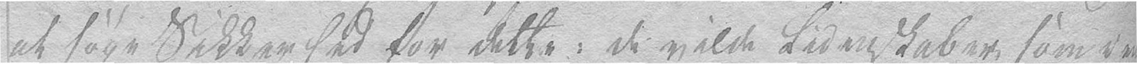at søge Sikkerhed for dette: de vilde Lidenskaber, som i en
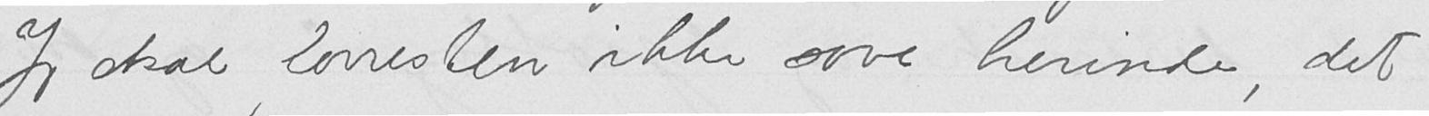Jeg skal forresten ikke sove herinde, det
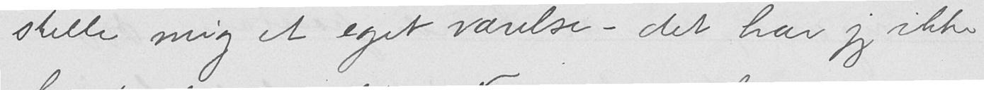stelle mig et eget værelse - det har jeg ikke
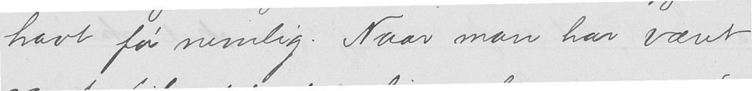havt før nemlig. Naar man har været
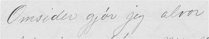Omsider gjør jeg alvor
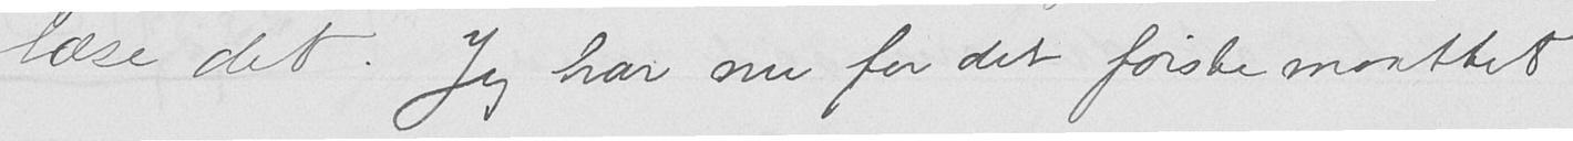læse det. Jeg har nu for det første maattet
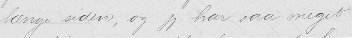længe siden, og jeg har saa meget
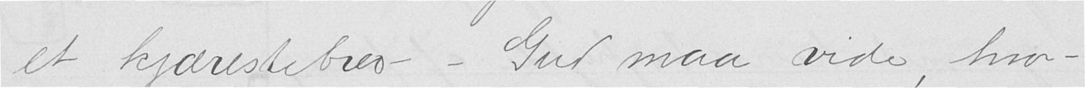et kjærestebrev - Gud maa vide, hvor-
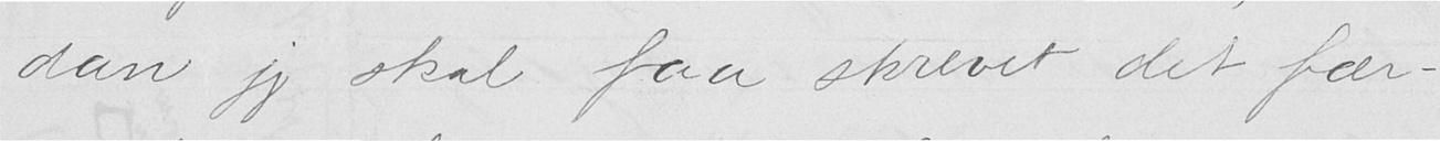dan jeg skal faa skrevet det fær-
